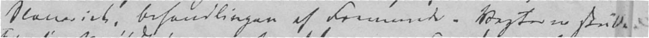Slaveriet, Behandlingen af Fremmede. Slægterne skulde
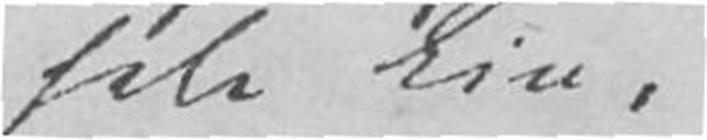hele Liv,
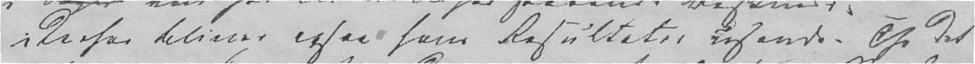Derfor bliver ogsaa hans Resultater usande. Thi det
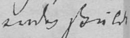endog skulde
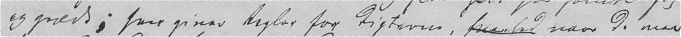og grædt; han giver Regler for Digterne, hvorled naar de maa
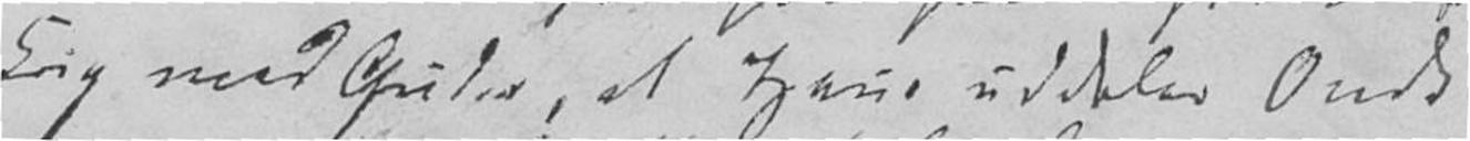Krig med Guder, at Zevs uddeler Ondt
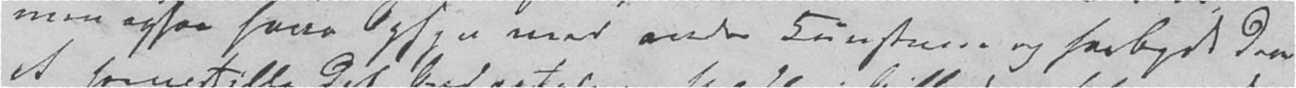men ogsaa have Opsyn med andre Kunstnere og forbyde dem
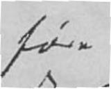føre
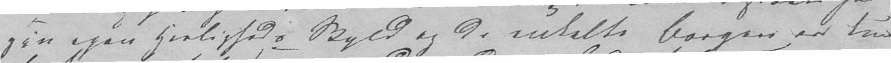sin egen Herligheds Skyld, og de enkelte Borgere er kun
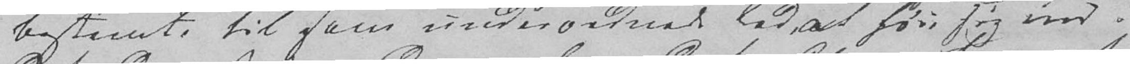bestemt til som underordnede Led at føie sig ind i
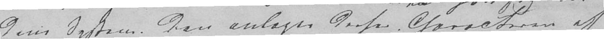dens System. Den antager derfor Characteren af
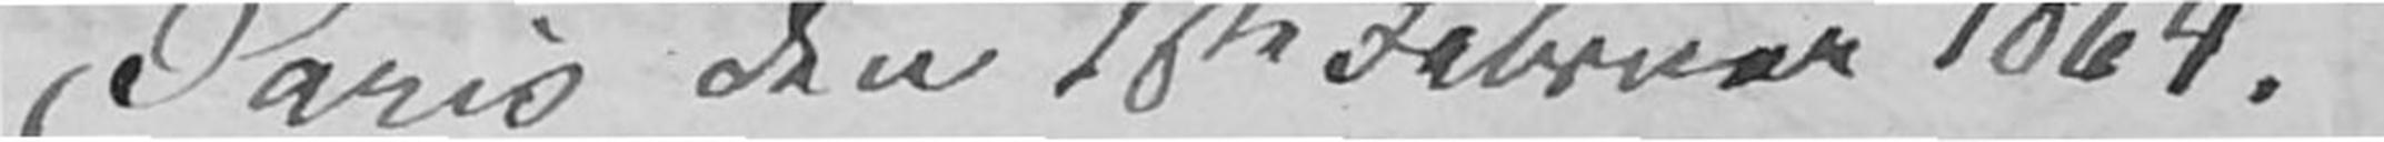Paris den 20de Februar 1864.
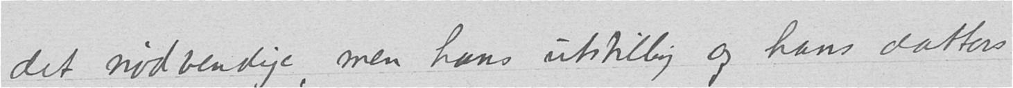det nødvendige, men hans utstilling og hans datters
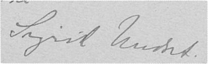Sigrid Undset.
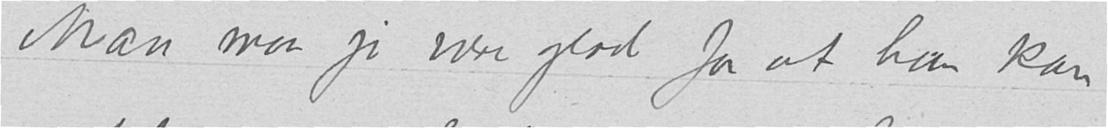Man maa jo være glad for at han kan
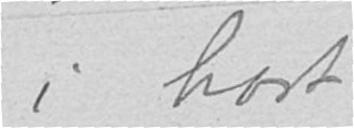i hast
